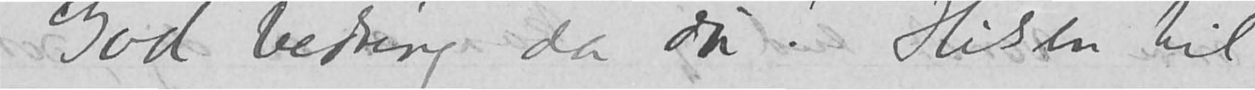God bedring da du! Hilsen til din
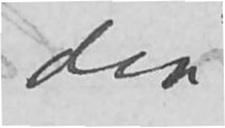din
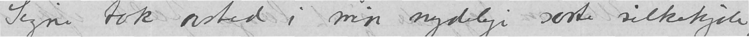Signe tok avsted i min nydelige sorte silkekjole,
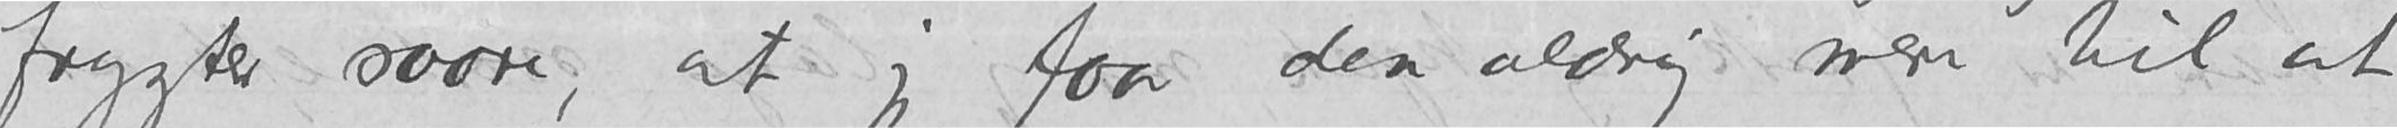frygter saare, at jeg faar den aldrig mere til at
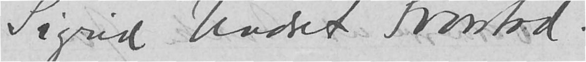Sigrid Undset Svarstad.
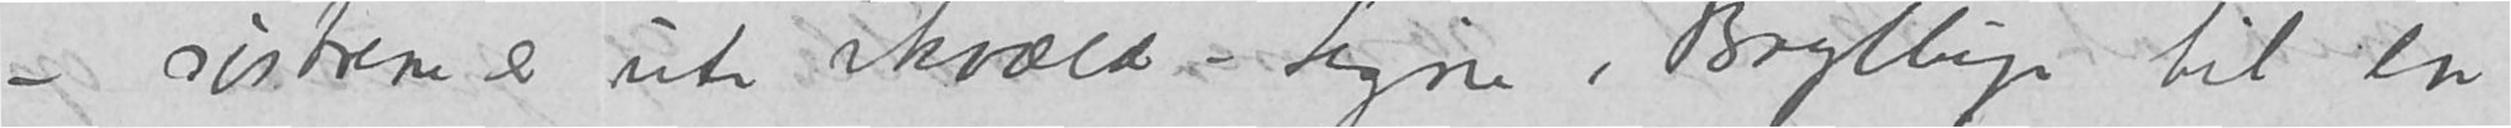– søstrene er ute ikvæld – Signe, Bryllup til en
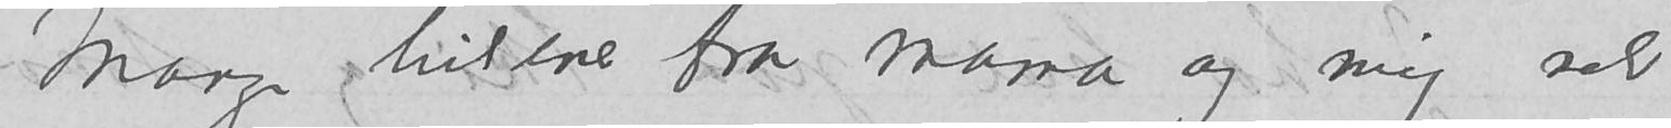Mange hilsener fra mama og mig selv
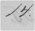14.
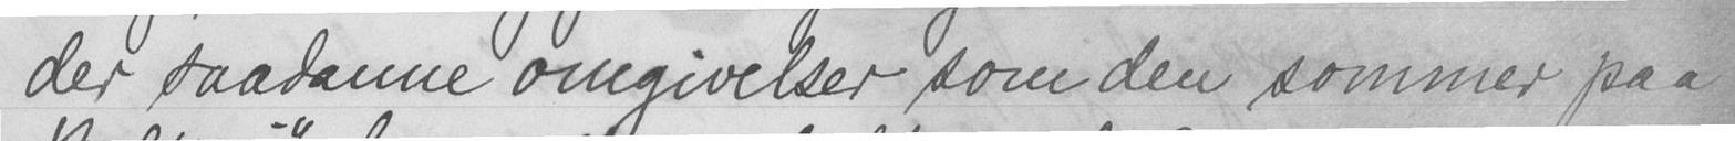der saadanne omgivelser som den sommer paa
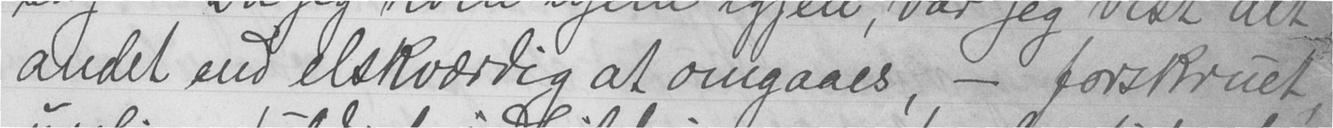andet end elskværdig at omgaaes, - forskruet,
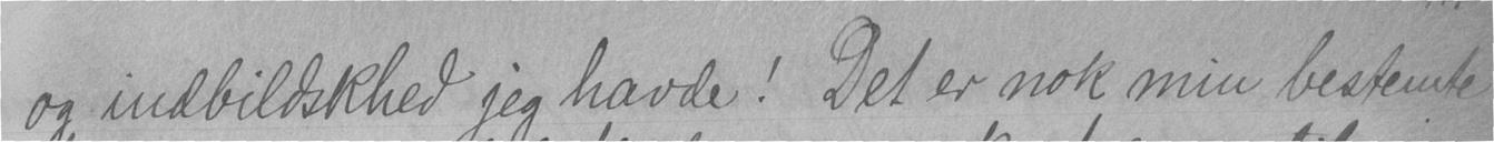og indbildskhed jeg havde! Det er nok min bestemte
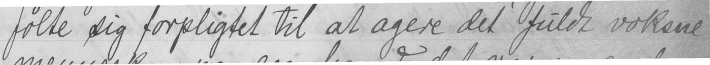følte sig forpligtet til at agere det fuldt voksne
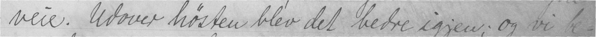veie. Udover høsten blev det bedre igjen; og vi be-
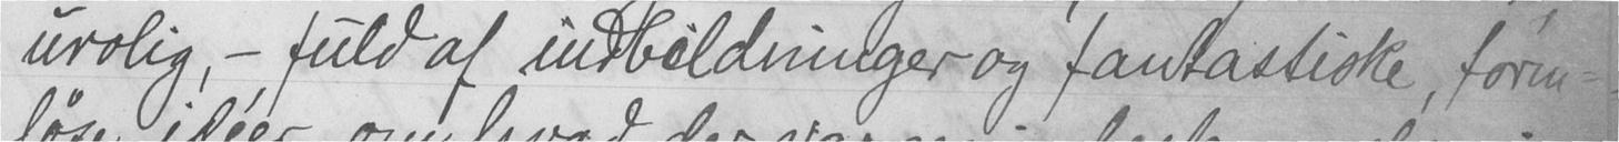urolig, - fuld af indbildninger og fantastiske, form-
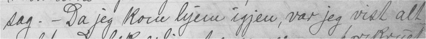sag. - Da jeg kom hjem igjen, var jeg vist alt
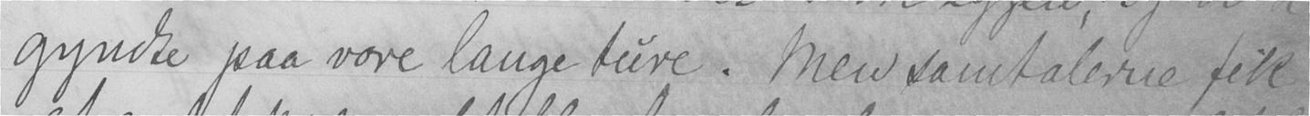gyndte paa vore lange ture. Men samtalerne fik
